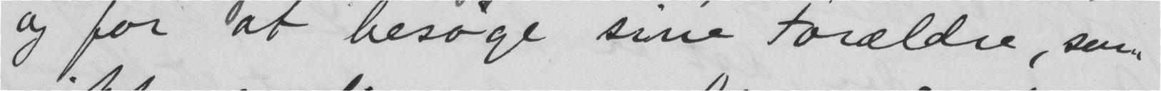og for at besøge sine Forældre, som
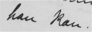han kan.
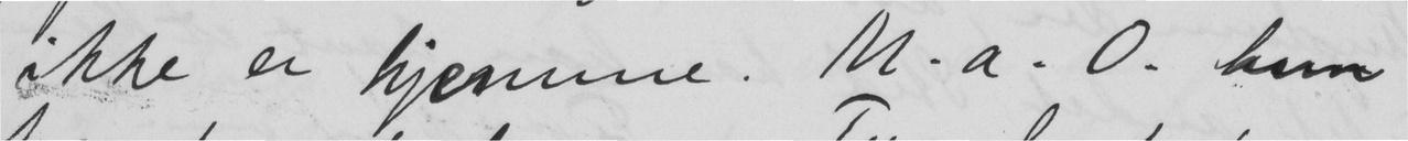ikke er hjemme. M.a. O. hun
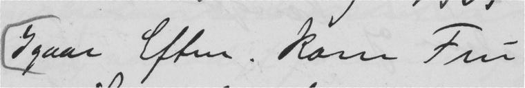Igaar Eften, kom Fru
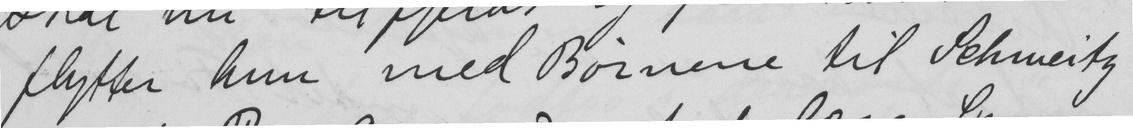flytter hun med Børnene til Schweitz,
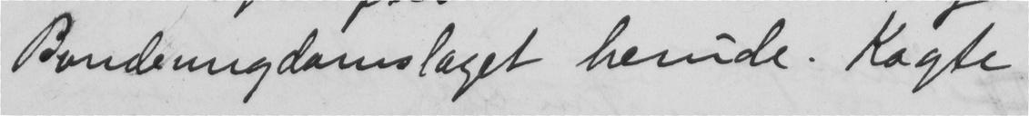Bondeungdomslaget herude. Kogte
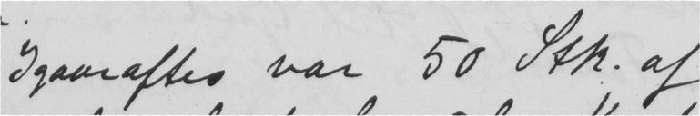Igaaraftes var 50 Stk. af
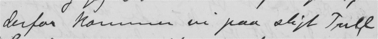derfor kommer vi paa sligt Tull
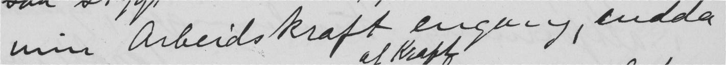min Arbeidskraft engang, endda
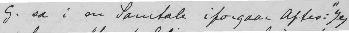G. sa i en Samtale iforgaar Aftes: "Jeg
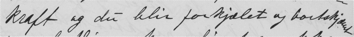Kraft og du blir forkjælet og bortskjæmt
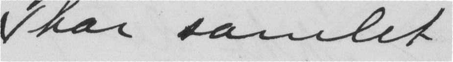har samlet
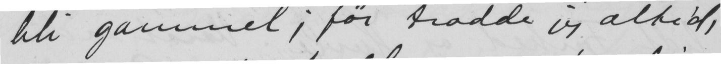bli gammel; før trodde jeg altid,
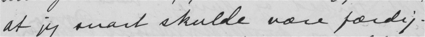at jeg snart skulde være færdig.
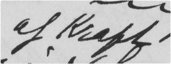af Kraft
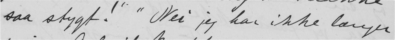saa stygt!" "Nei jeg har ikke længer
